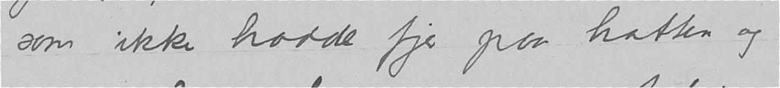som ikke hadde fjer paa hatten og
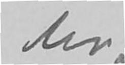din
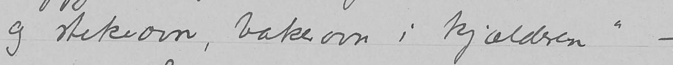og stekeovn, bakeovn i kjælderen» –
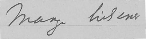Mange hilsener
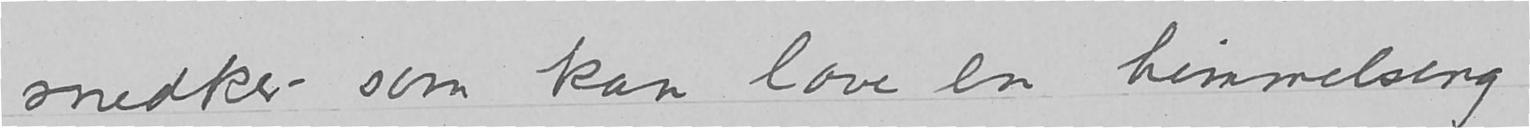snedker som kan lave en himmelseng
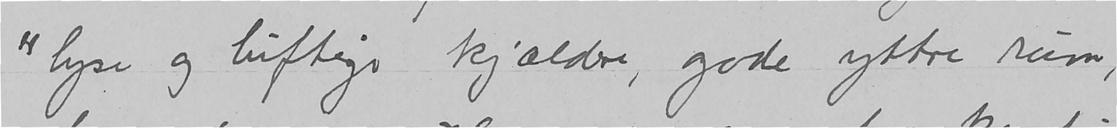«lyse og luftige kjældere, gode yttre rum,
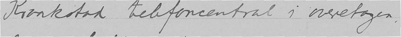Kraakstad telefoncentral i overetagen,
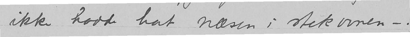ikke hadde hat næsen i stekovnen –.
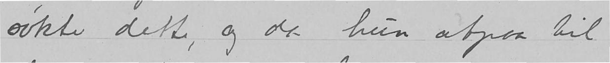søkte dette, og da hun atpaa til
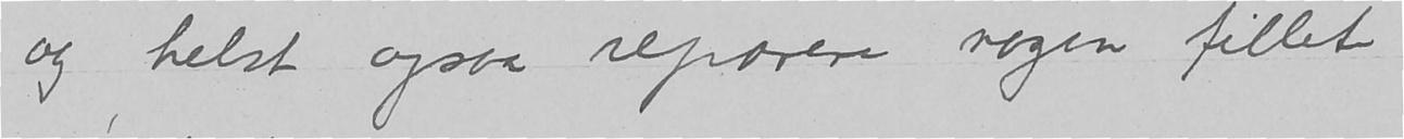og helst ogsaa reparere nogen fillete
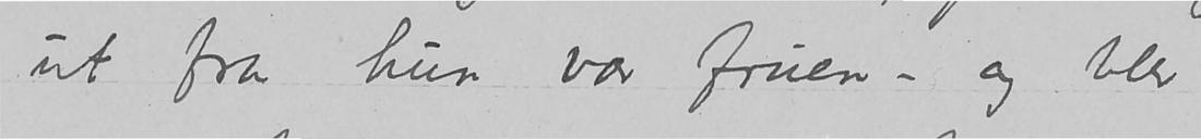ut fra hun var fruen – og blev
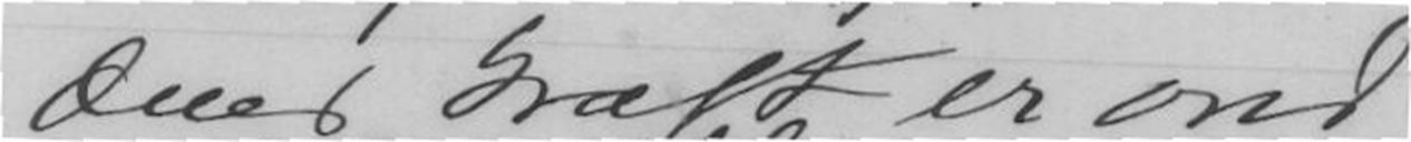dens kraft er ond,
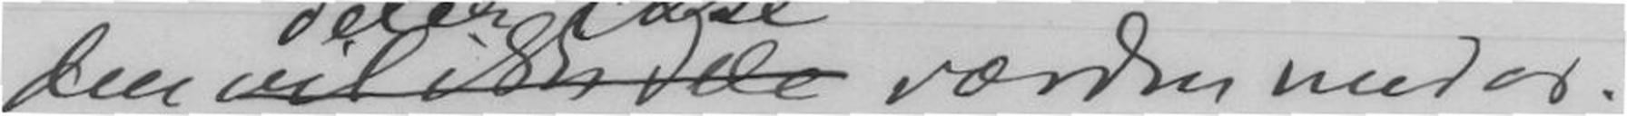den deler ikke vil ikke Dele værden med os.
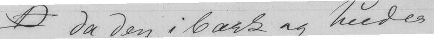1) da den i bark og huder
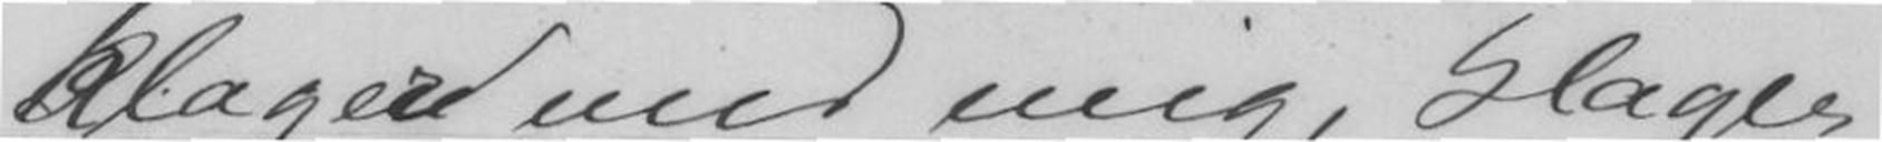klager med mig, klager
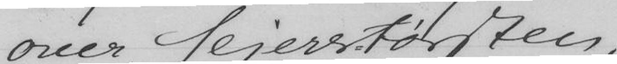over Sejerstørsten,
